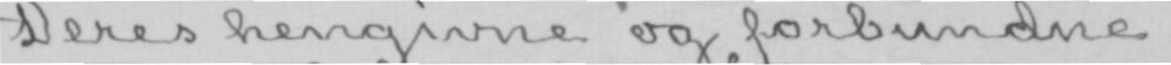Deres hengivne og forbundne
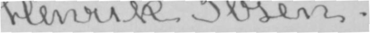Henrik Ibsen.
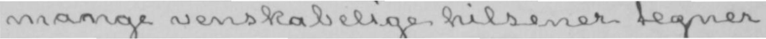mange venskabelige hilsener tegner
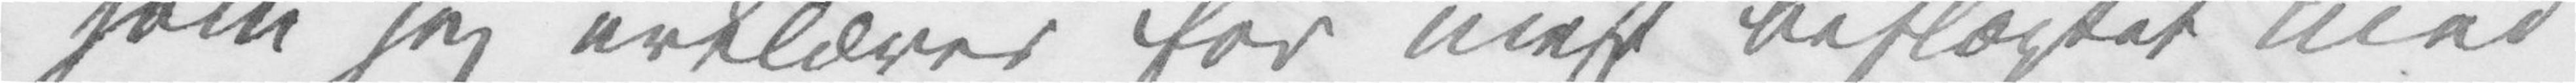som jeg erklærer for mest beslægtet med
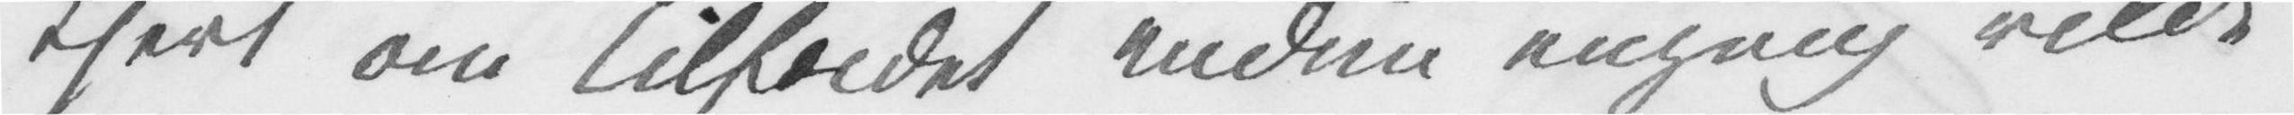kjert om Tilfældet endnu engang vilde
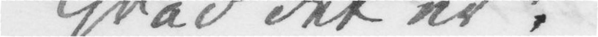hvad det er.
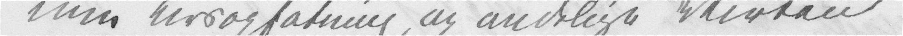min Livsopfatning og åndelige Virken
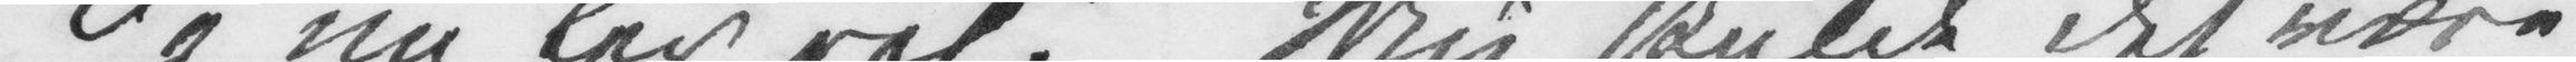Og nu lev vel! Mig skulde det være
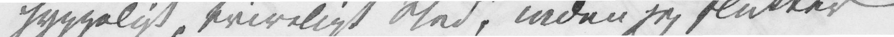hyggeligt, triveligt Sted, inden jeg slutter
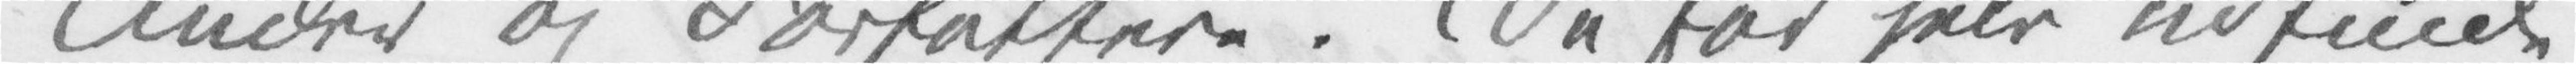Ånder og Forfattere. De får selv udfinde
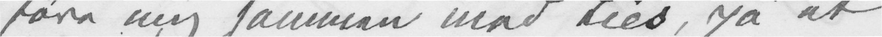føre mig sammen med Lies, på et
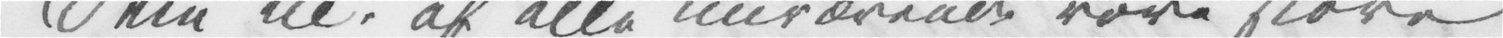Dem Lie, af alle nuværende vore store
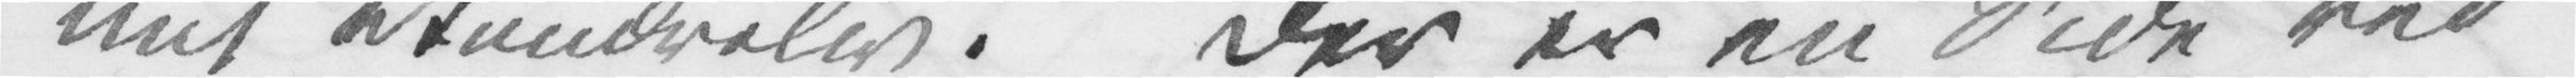mit Vandreliv. Der er en Side ved
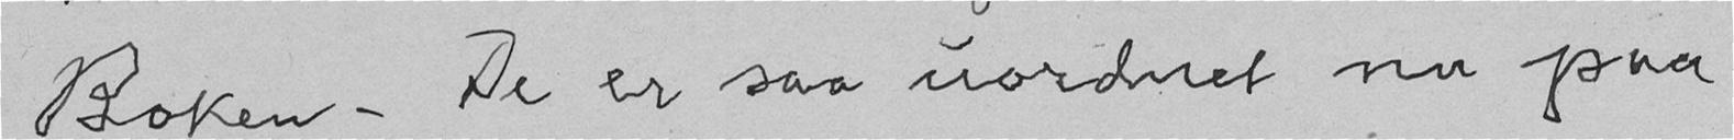Boken. De er saa uordnet nu paa
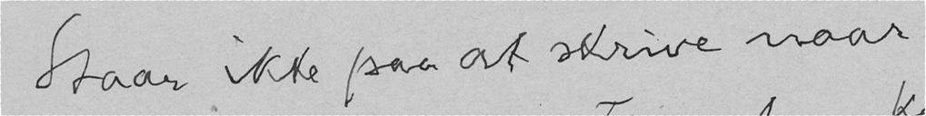Staar ikke paa at skrive naar
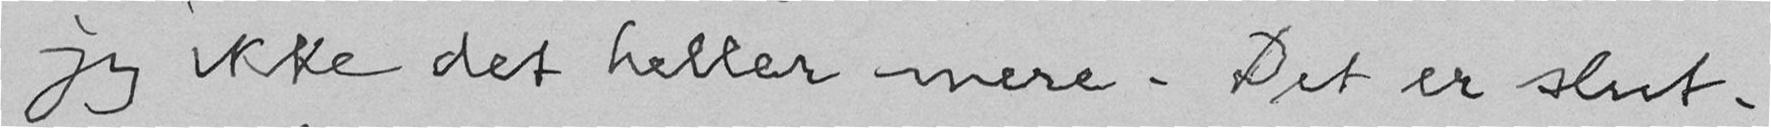jeg ikke det heller mere. Det er slut.
